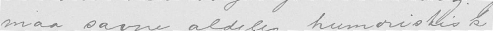maa savne aldeles humoristisk
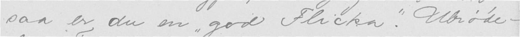saa er du en "god Flicka". Ubrøde-
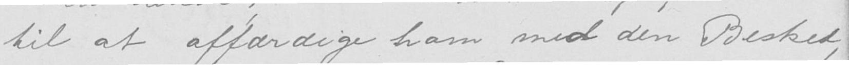til at affærdige ham med den Besked,
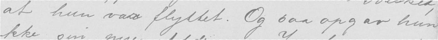at hun var flyttet. Og saa oppgav hun
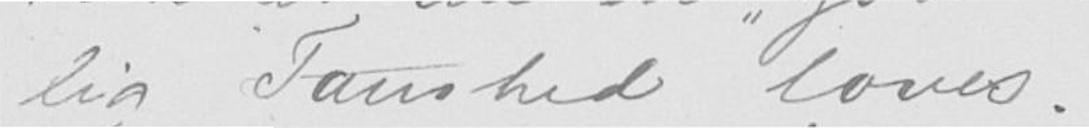lig Taushed loves.
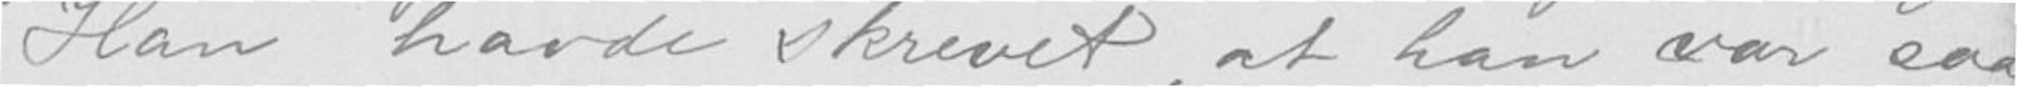Han havde skrevet, at han var saa
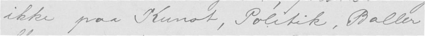ikke paa Kunst, Politik, Baller
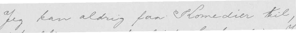Jeg kan aldrig faa Komedier til,
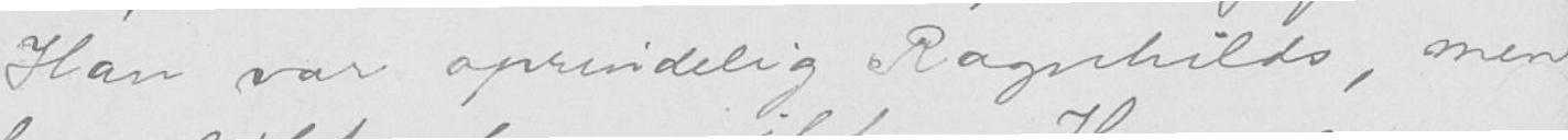Han var oprindelig Ragnhilds, men
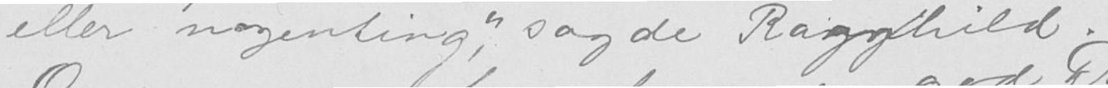eller nogenting", sagde Ragnhild.
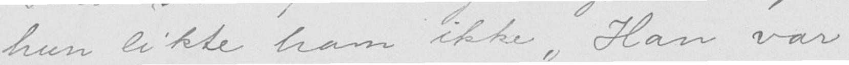hun likte ham ikke, "Han var
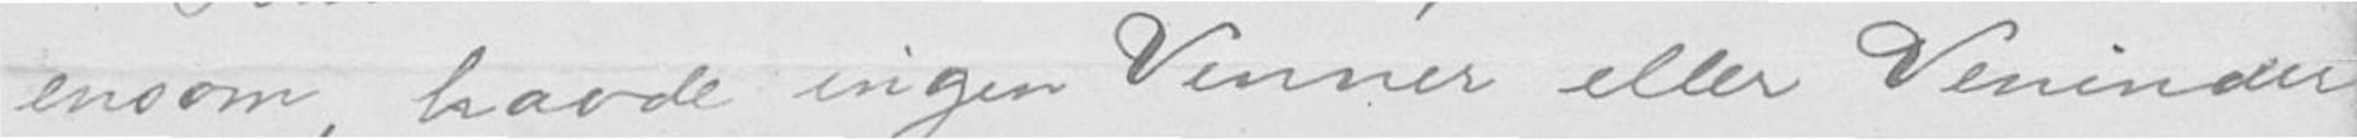ensom, havde ingen Venner eller Veninder
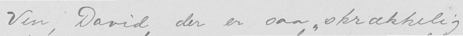Ven, David, der er saa "skrækkelig
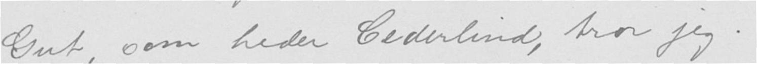Gut, som heder Cederlind, tror jeg.
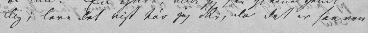Dig; love det vist tør jeg ikke, da det er saa van
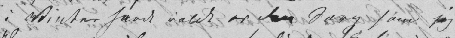i Vinter havde voldt os den Sorg som jeg
